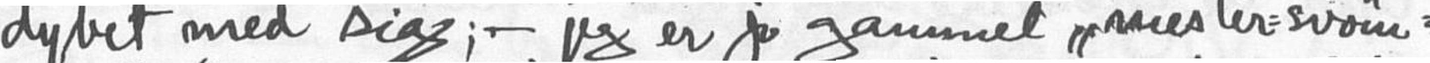dybet med sig ; - jeg er jo gammel "mester-svøm-
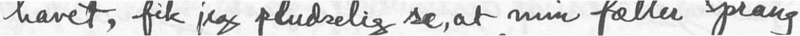havet, fik jeg pludselig se, at min fætter sprang
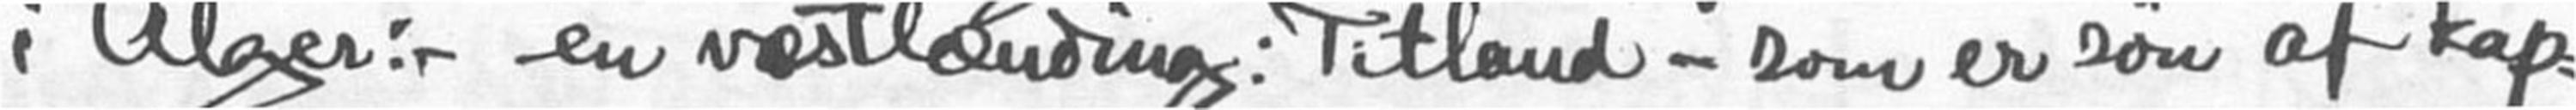i Alger: - en væstlænding: Titland - som er søn af kap-
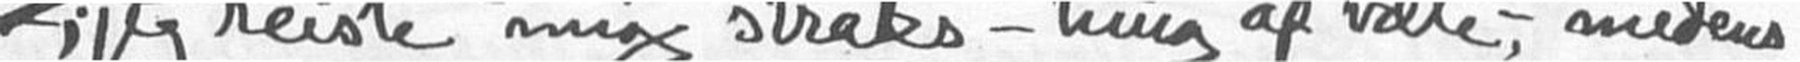; jeg vilde reise mig straks medens
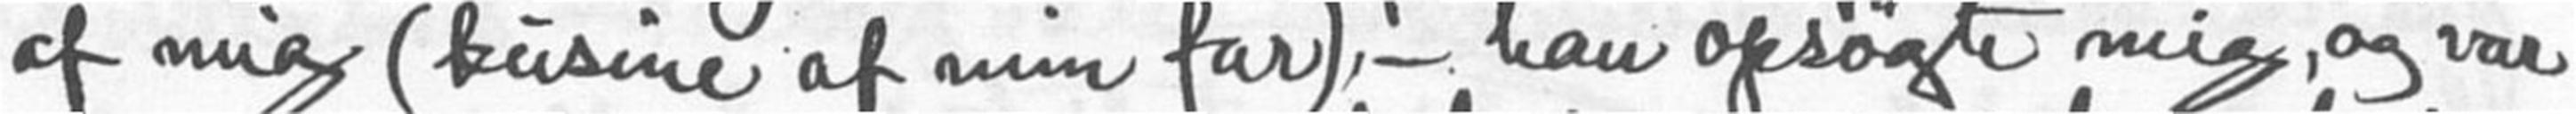af mig (kusine af min far), - han opsøgte mig, og var
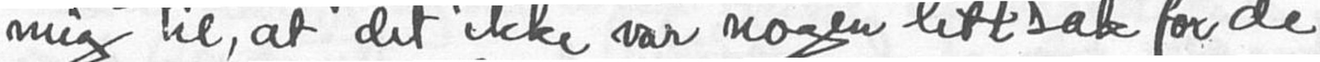mig til, at det ikke var nogen lett sak for de
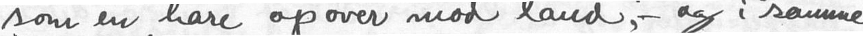som en hare opover mod land, - og i samme
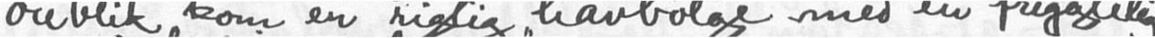øieblik kom en rigtig "havbølge" med en frygtelig
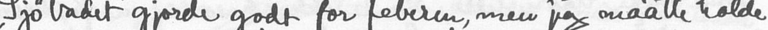"Sjøbadet" gjorde godt for feberen, men jeg maatte holde
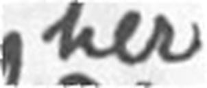her
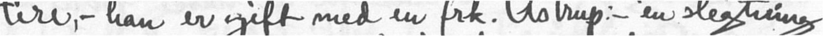tere, - han er gift med en frk. Astrup:- en slegtning
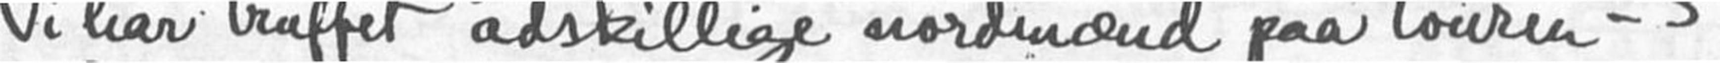Vi har truffet adskillige nordmænd paa touren - 3
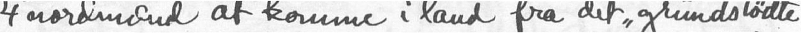4 nordmænd at komme i land fra det "grundstødte"
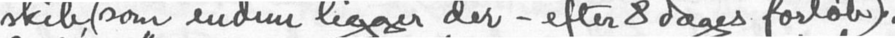skib (som endnu ligger der - efter 8 dages forløb).
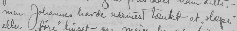men Johannes havde nærmest tænkt at "slæpe"
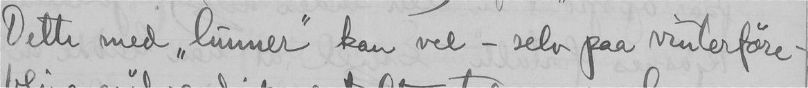Dette med "lunner" kan vel - selv paa vinterføre -
